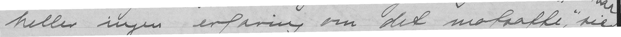heller ingen erfaring om det motsatte, sier
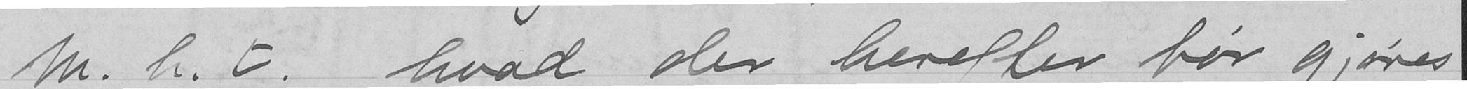M.h.t. hvad der herefter bør gjøres
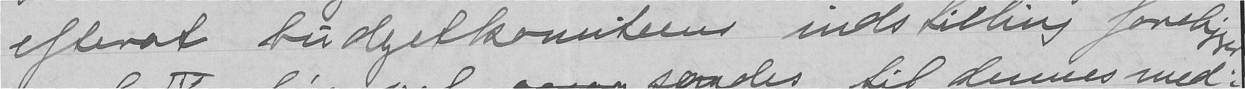efterat budgetkomiteens indstilling foreligger
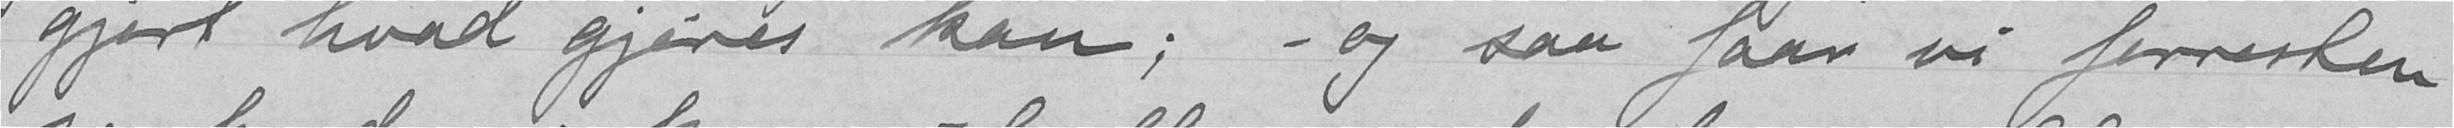gjort hvad gjøres kan; - og saa faar vi forresten
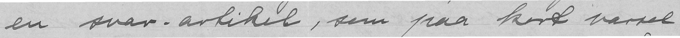en svar-artikel, som paa kort varsel
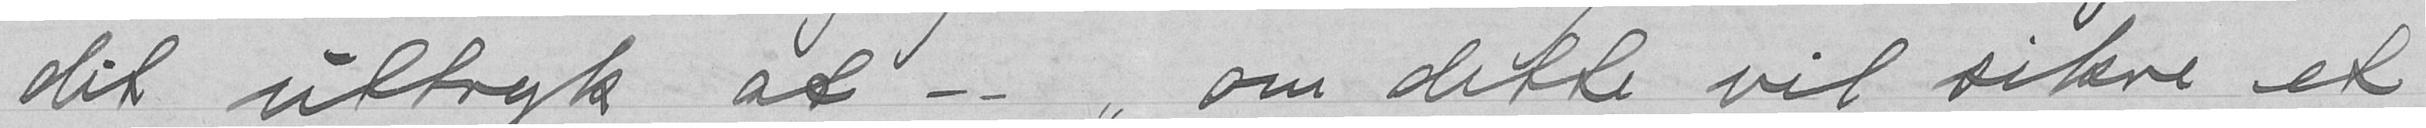det uttryk at - om dette vil sikre et
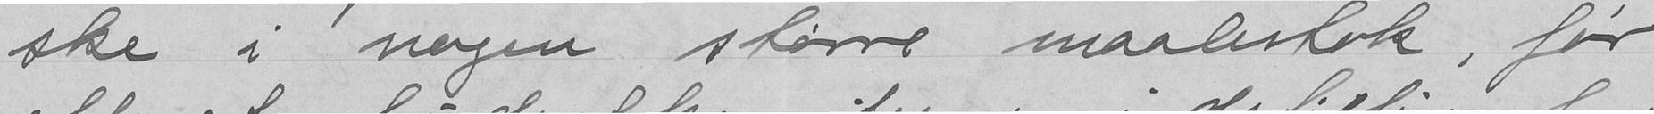ske i nogen større maalestok, før
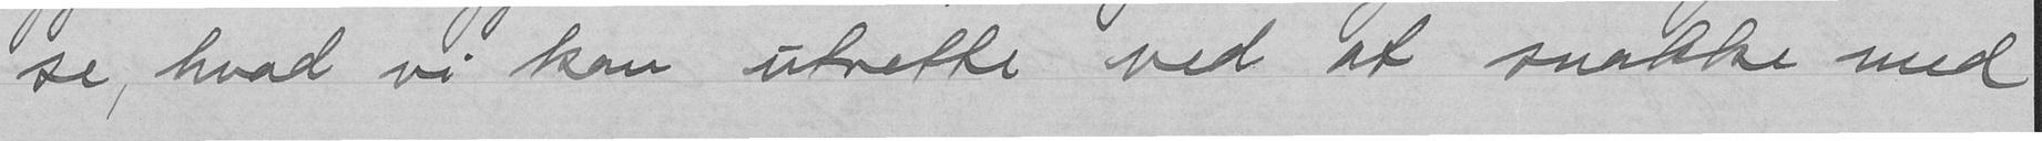se, hvad vi kan utrette ved at snakke med
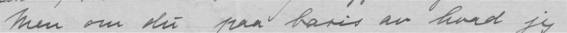Men om du paa basis av hvad jeg
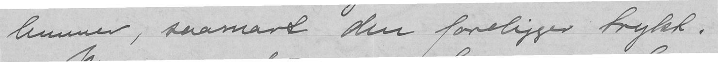lemmer, saasnart den foreligger trykt.
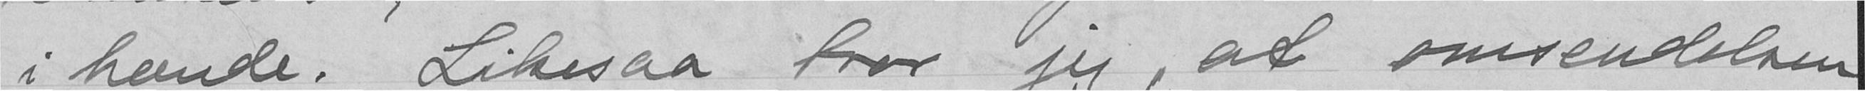i hende. Likesaa tror jeg, at omsendelsen
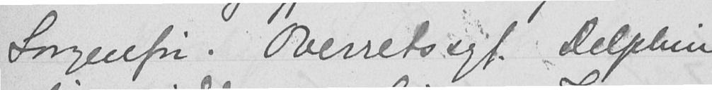Sorgenfri. Overretssagf. Delphin
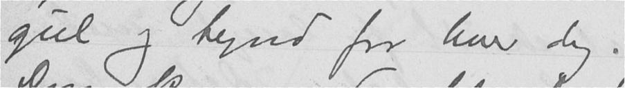gul og tynd for hver dag.
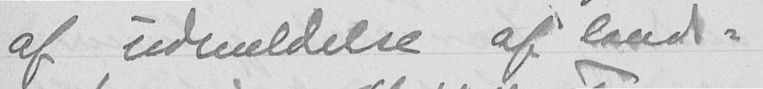af udmeldelse af lands-
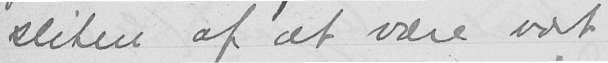sliten af at være vært
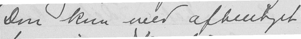Don kom med aftentoget
